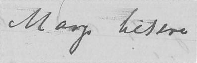Mange hilsener
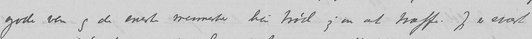gode ven og de eneste mennesker han brød sig om at træffe. Jeg er svært
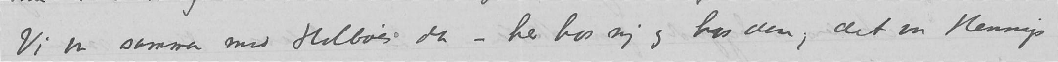Vi en sammen med Holbøes da – her hos mig og hos den, det var Hennigs
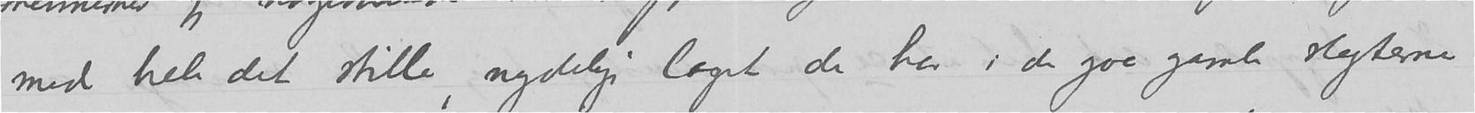med hele det stille, nydelige laget de har i de goe gamle slegterne
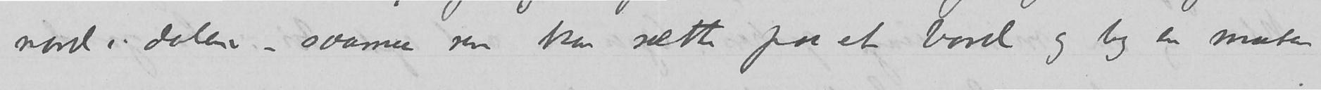nord i dalene – saanne som kan sætte paa et bord og by en maten
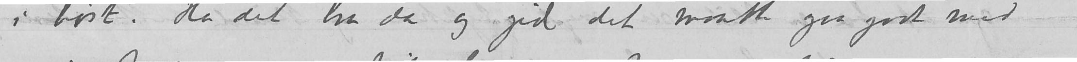i høst. Ha det bra da og gid det maatte gaa godt med
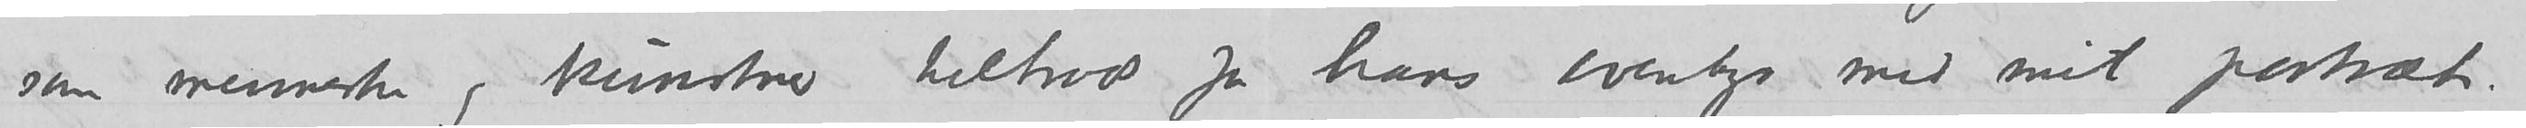som menneske og kunstner tiltrods for hans eventyr med mit portræt.
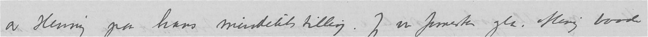av Hennig paa hans mindeutstilling. Jeg er forresten gla i Hennig både
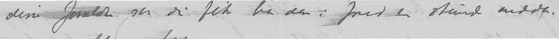dine forældre saa du fik ha den i fred stund endda.
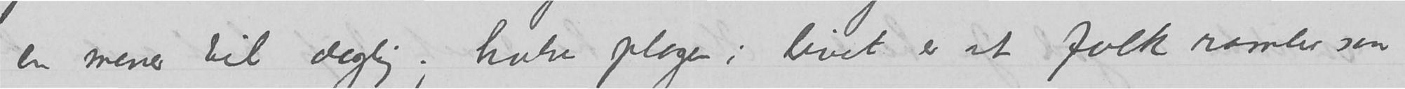en mener til daglig; halve plagen i livet er at folk ramler saa
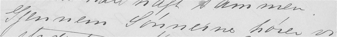Gjennem Sønnerne hører vi
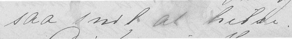saa snil at hilse.
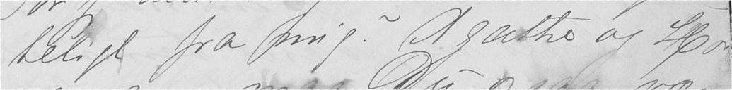teligt fra mig? Agathe og Hor-
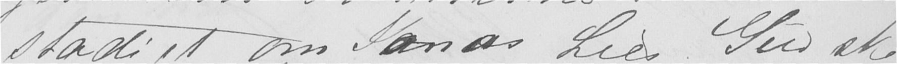stadigt om Jonas Lies. Gud ske
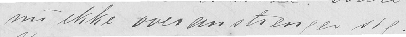nu ikke overanstrenger sig.
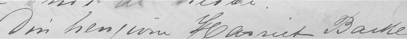Din hengivne Harriet Backer
